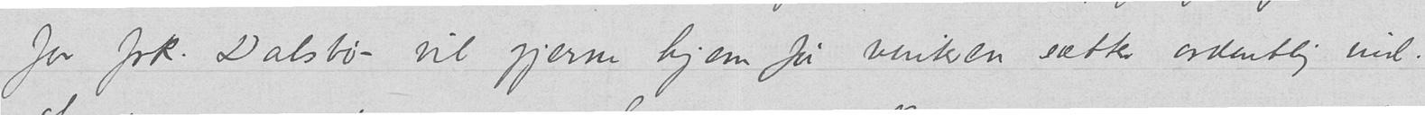for frk. Dalsbø vil gjerne hjem før vinteren sætter ordentlig ind.
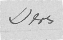Deres
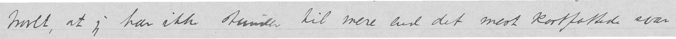travlt, at jeg har ikke stunder til mere end det mest kortfattede svar.
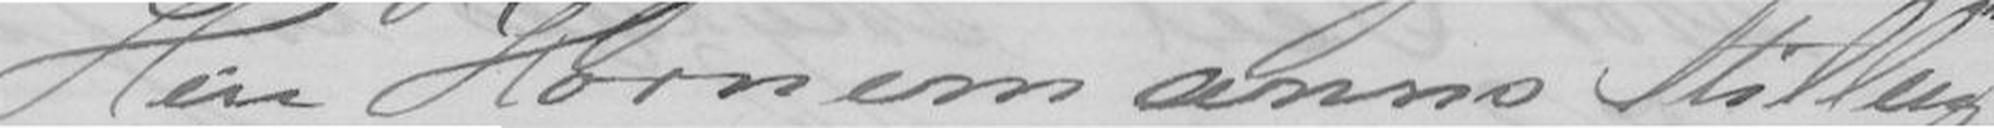Herr Hornum anno Stilling
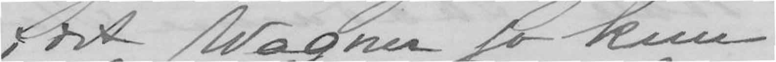i det Wagner jo kun
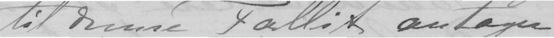til denne Fallit antager
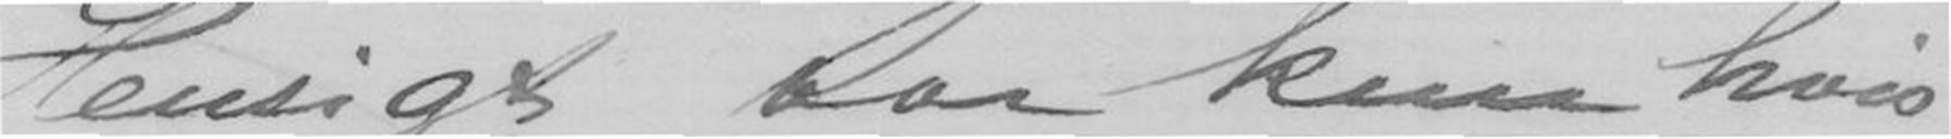Hensigt var kun hvis
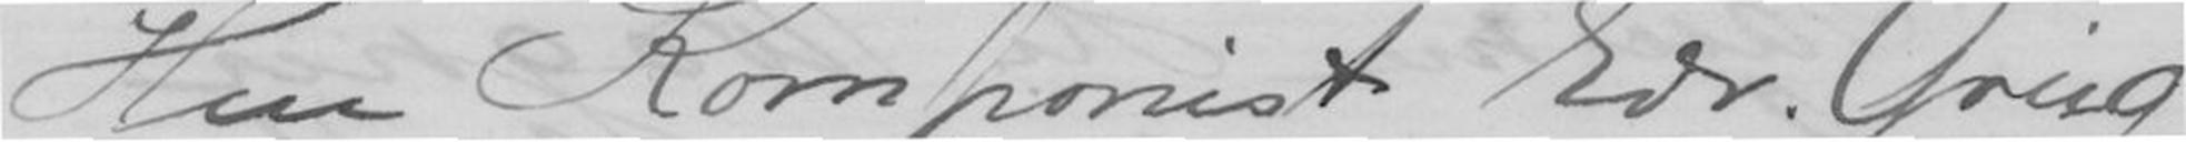Herr Komponist Edv. Grieg
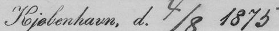Kjøbenhavn, d.4/8 1875
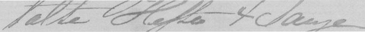talte Heftes - 4 Sangen
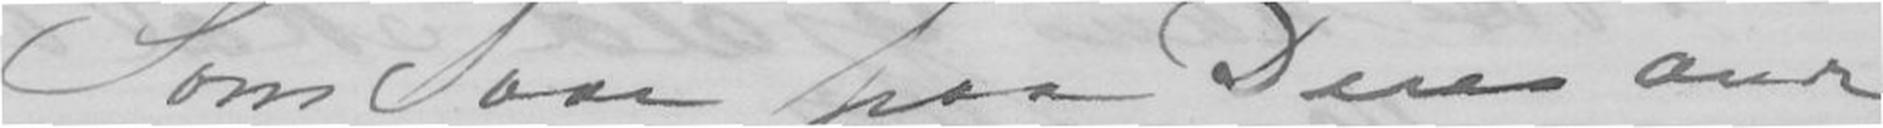Som Svar paa Deres andre
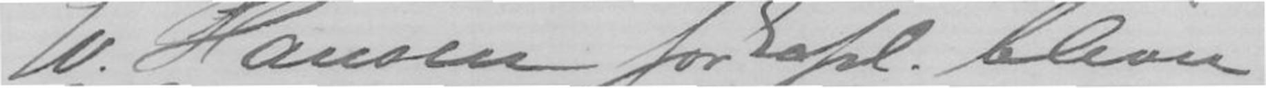Hr Hansen for Expl. bliver
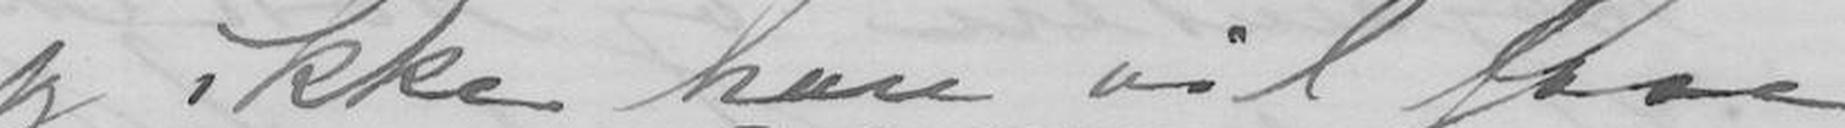jeg ikke han vil faae
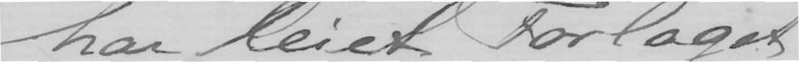har leiet Forlaget
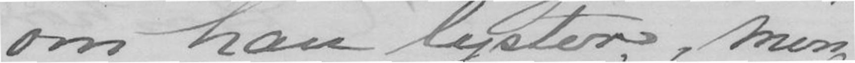om han lyster, min
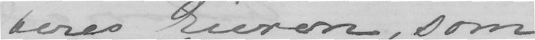veres Eieren, som
